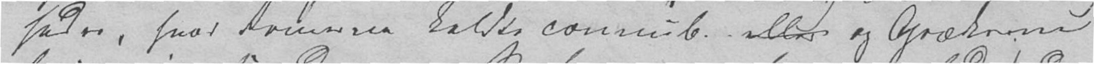heder, hvad Romerne kaldte connub. eller og Grækerne
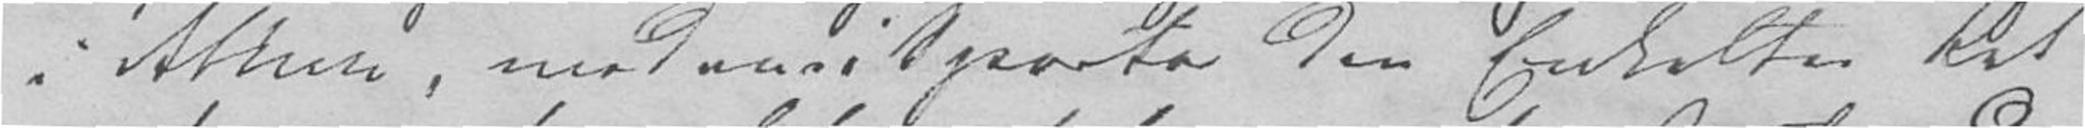i Athen, medens i Sparta den Enkeltes Ret
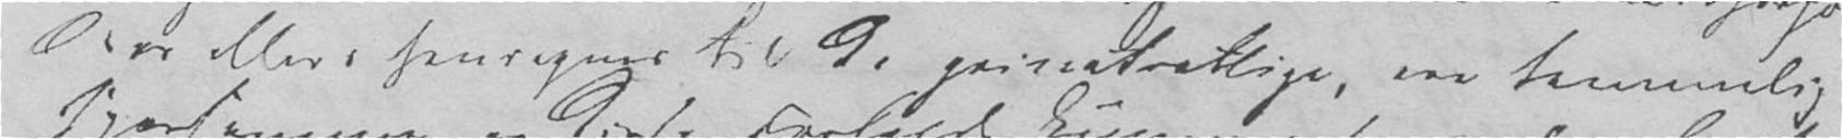der ellers henregnes til de privatretlige, ere temmelig
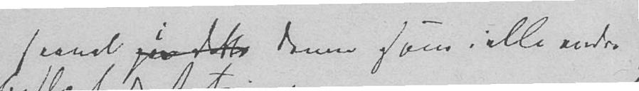saavel i denne som i alle andre
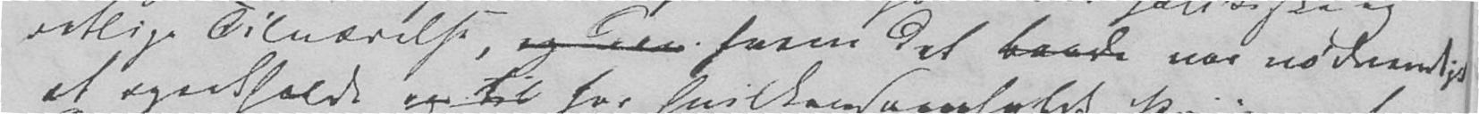retlige Tilværelse, og den hvem det baade var nødvendigt
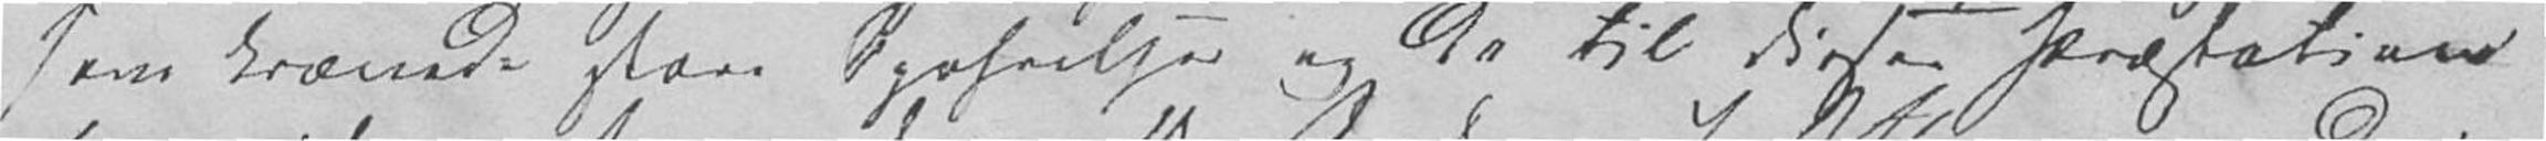som krævede store Opofrelser og de til disse Præstationer
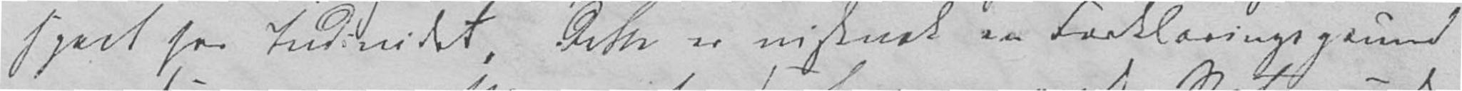spect for Individet. Dette er vistnok en Forklaringsgrund
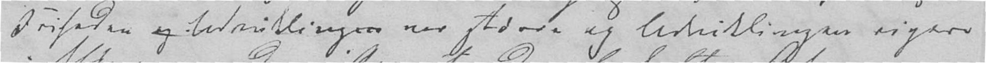Friheden og Udviklingen var større og Udviklingen rigere
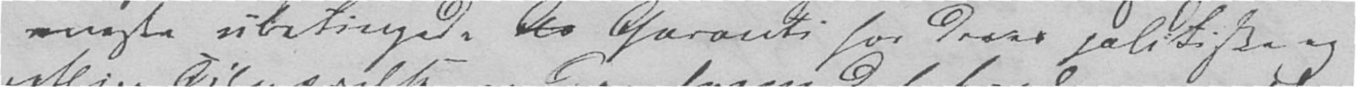eneste ubetingede Ro Garanti for deres politiske og
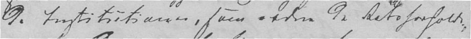de Institutioner, som ordne de Retsforholde,
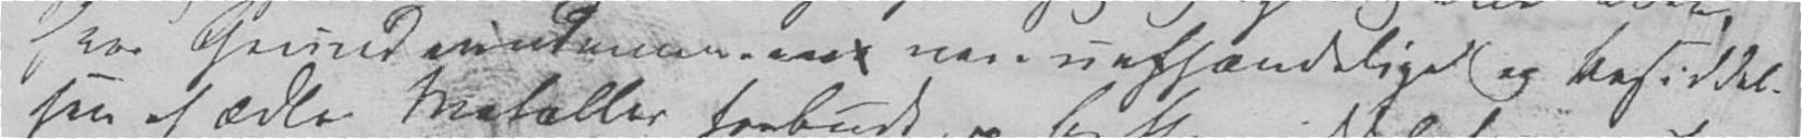hvor Grundeiendommene vare uafhændelige og Besiddel-
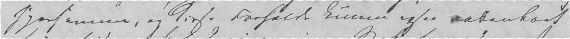sparsomme, og disse Forholde kunne ogsaa aabenbart
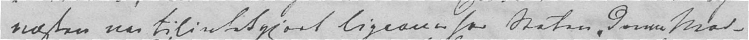næsten var tilintetgjort ligeoverfor Staten. Denne Mod-
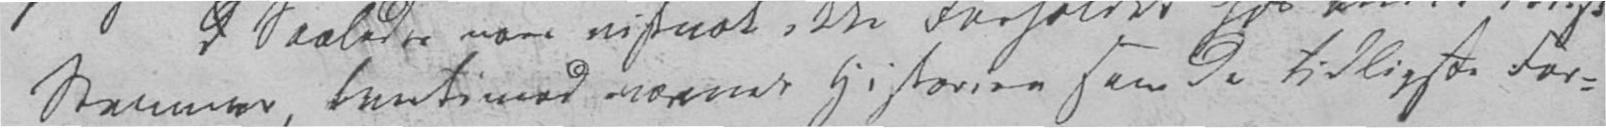Stammer, tvertimod nævner Historien som de tidligste For-
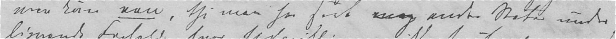men kun een, thi man har seet meg andre Stater under
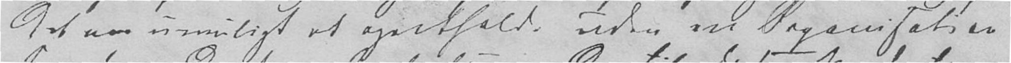det var umuligt at opretholde uden en Organisation
